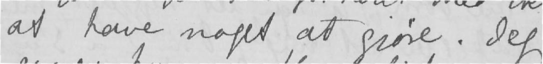at have noget at gjøre. Jeg
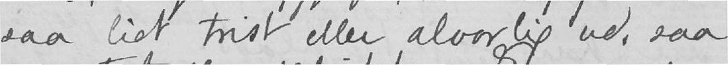saa lidt trist eller alvorlig ud, saa
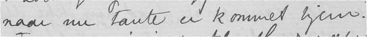naar nu tante er kommet hjem.
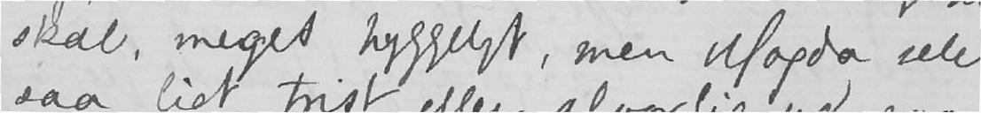skab, meget hyggeligt, men Magda selv
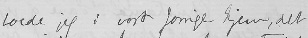boede jeg i vort forrige hjem, det
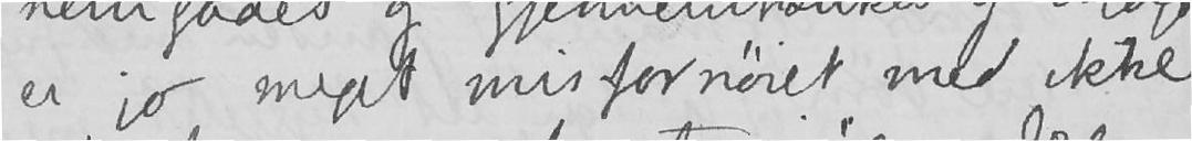er jo meget misfornøiet med ikke
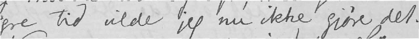gre tid vilde jeg nu ikke gjøre det.
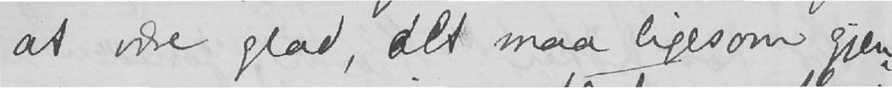at være glad, det maa ligesom gjen-
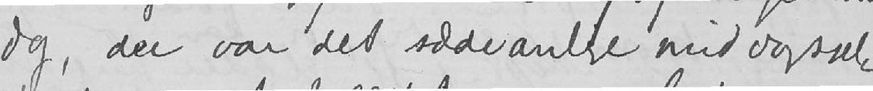dag, der var det sædvanlige middagssel-
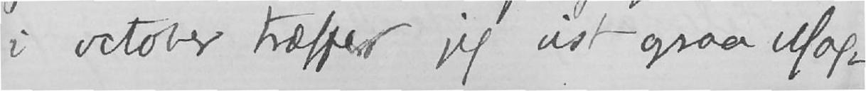i october træffer jeg vist ogsaa Mag-
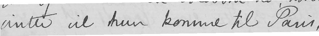vinter vil hun komme til Paris,
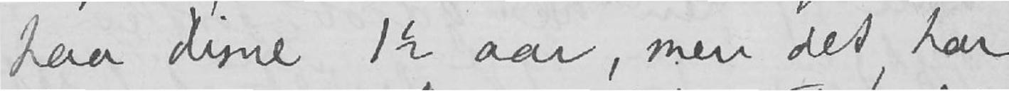paa disse 1½ aar, men det har
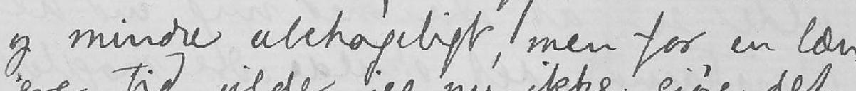og mindre ubehageligt, men for en læn-
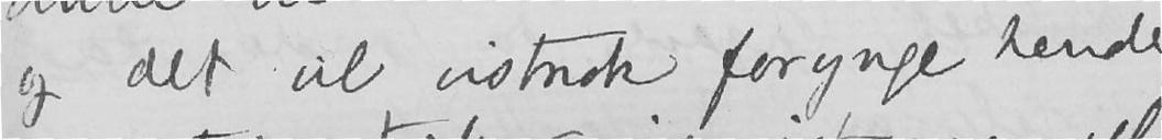og det vil vistnok forynge hende,
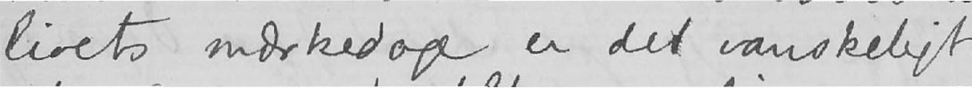livets mærkedage er det vanskeligt
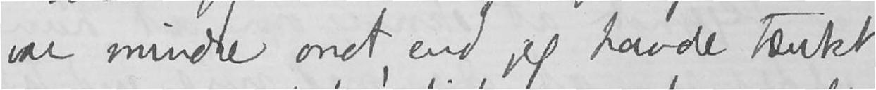var mindret ondt, end jeg havde tænkt
